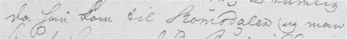da han kom til Romsdalen og man
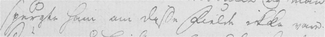spurgte ham om disse Fielde ikke vare
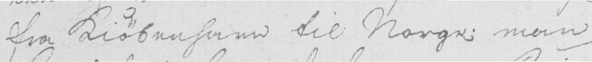fra Kiøbenhavn til Norge; man
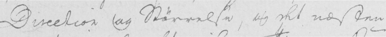Direction og Størrelse, og det næsten
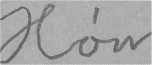Høn
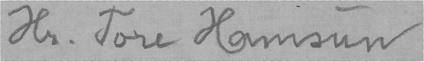Hr. Tore Hamsun
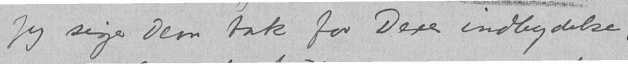Jeg siger Dem tak for Dere indbydelse,
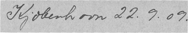Kjøbenhavn 22.9.09.
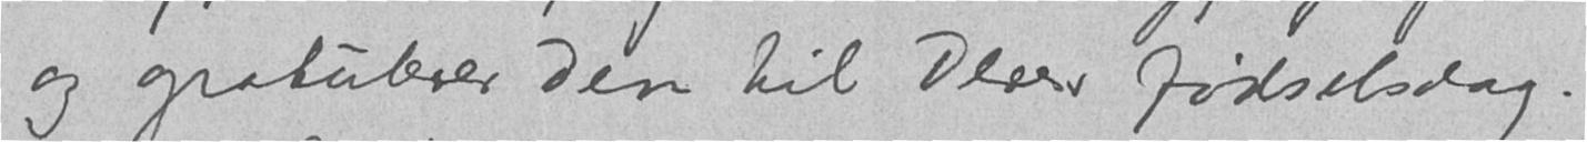og gratulerer Dem til Deres fødselsdag.
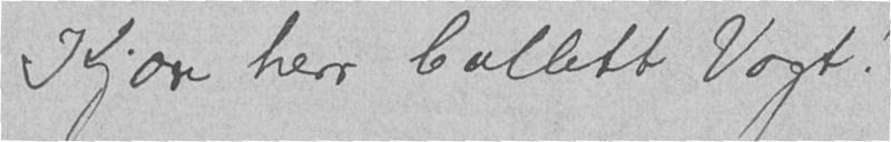Kjære herr Collett Vogt!
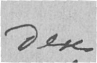Deres
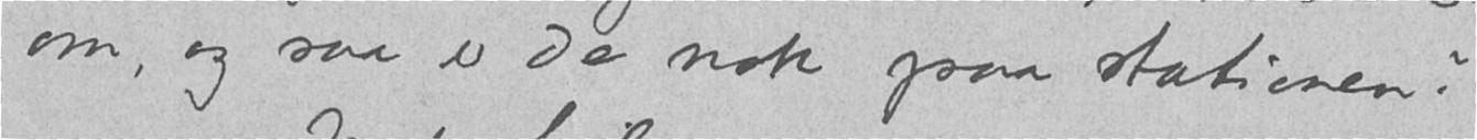om, og saa er De nok paa stationen?
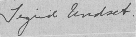Sigrid Undset.
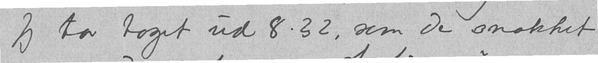Jeg tar toget ud 8.32, som De snakket
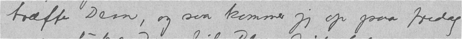træffe Dem, og saa kommer jeg op paa fredag
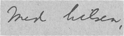Med hilsen,
